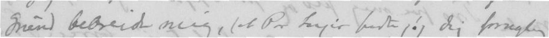Grund bebreide mig, (et Par Linjer sendte jeg dig forsegle
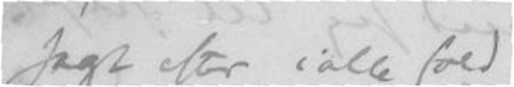Jagt efter. iallefald
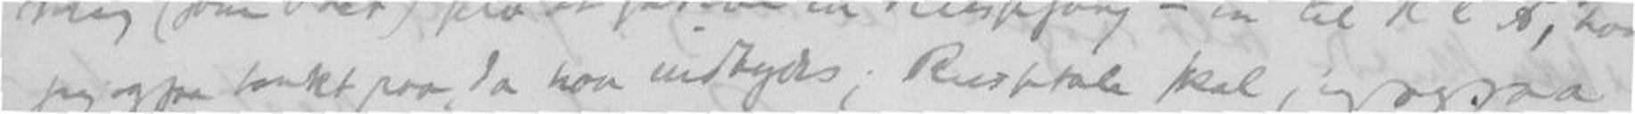jeg ogsaa tænkt paa, da han indbydes; Pressetale skal jeg ogsaa
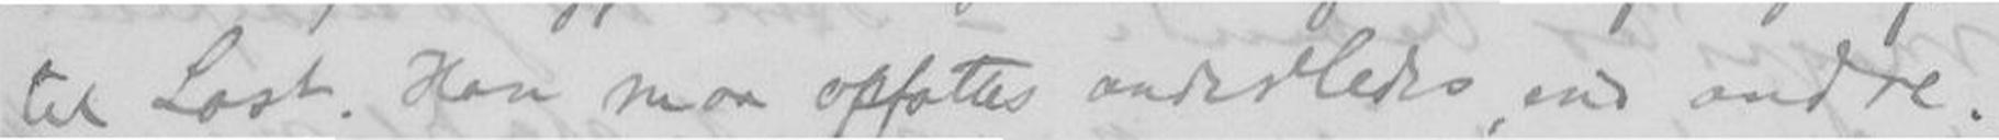til Last. Han maa opfattes anderledes end andre.
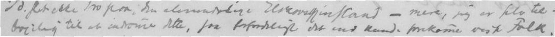bøjelig til at indrøme dette, saa forfærdeligt det end kunde forekome vist Folk.
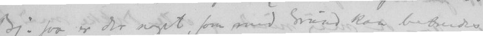Bj. saa er der noget som med grund kan bebreides
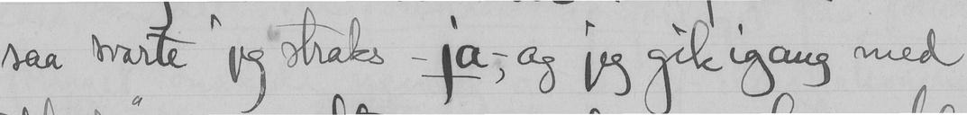saa svarte jeg straks - ja, - og jeg gik igang med
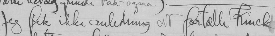Jeg fik ikke anledning at fortælle Kinck
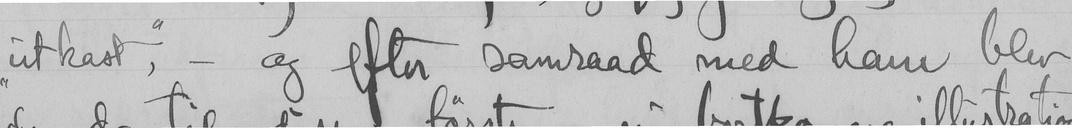"utkast", - og efter samraad med ham blev
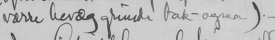værre bevæg grunde bak - ogsaa). -
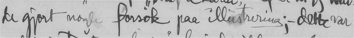de gjort nogle forsøk paa illustrering; - dette var
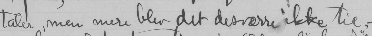taler, men mere blev det desværre ikke til, -
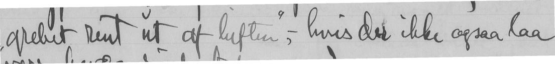"grebet rent ut af luften, - hvis der ikke ogsaa laa
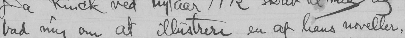bad mig om at illustrere en af hans noveller,
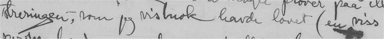streringen, som jeg vistnok havde lovet (en viss
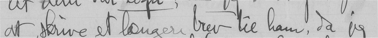at skrive et længere brev til ham, da jeg
